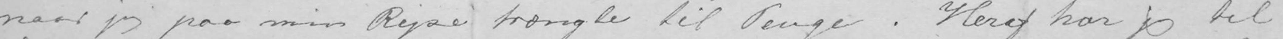naar jeg paa min Rejse trængte til Penge. Heraf har jeg til
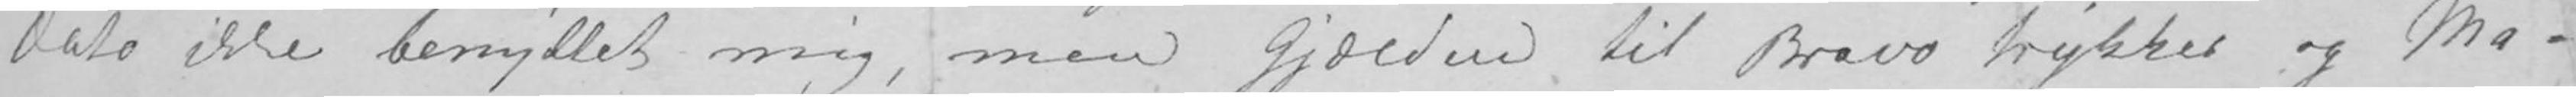Dato ikke benyttet mig, men Gjælden til Bravo trykker og Ma-
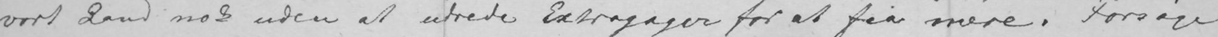vort Land nok uden at udrede Extragager for at faa mere. Forsøge
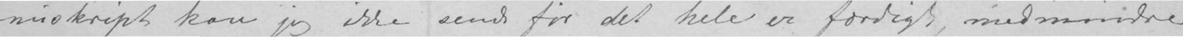nuskript kan jeg ikke sende før det hele er færdigt, medmindre
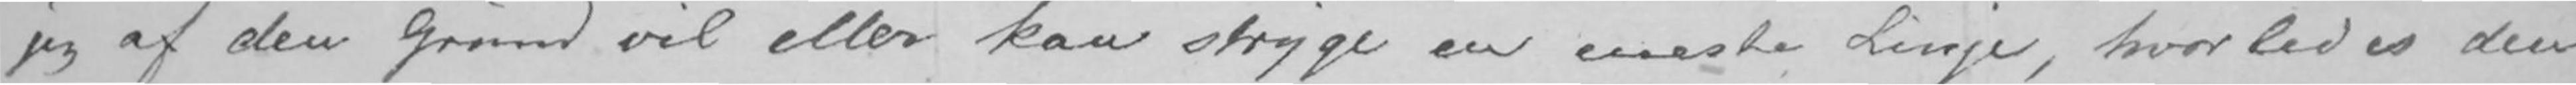jeg af den Grund vil eller kan stryge en eneste Linje, hvorledes den
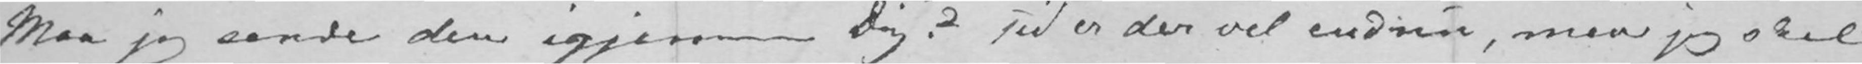Maa jeg sende den igjennem Dig? Tid er der vel endnu, men jeg skal
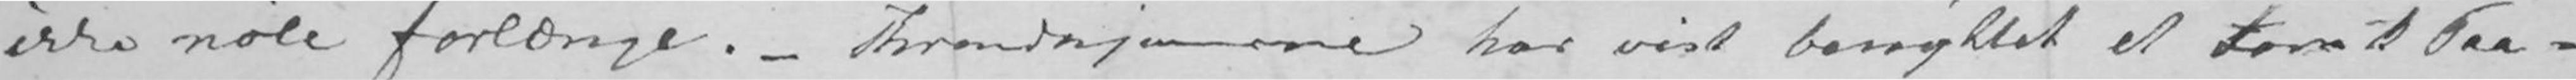ikke nøle forlænge.- Throndhjemmerne har vist benyttet et tomt Paa-
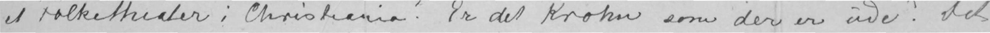et Folketheater i Christiania? Er det Krohn som der er ude? Det
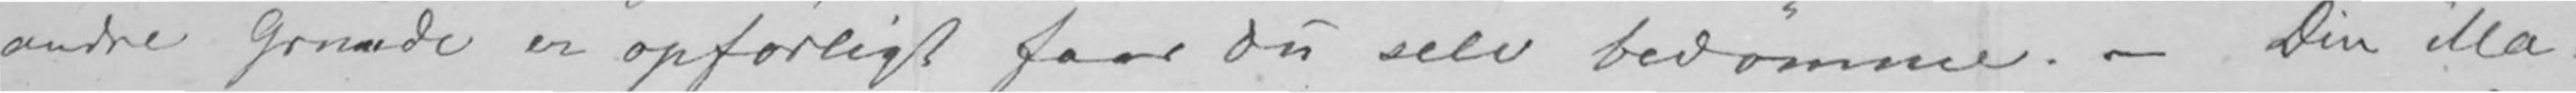andre Grunde er opførligt faar Du selv bedømme.- Din «Ma-
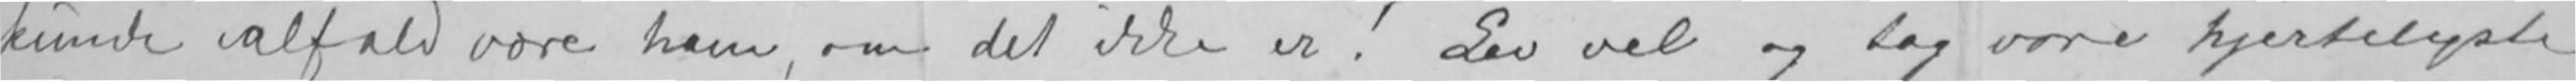kunde ialfald være ham, om det ikke er! Lev vel og tag vore hjerteligste
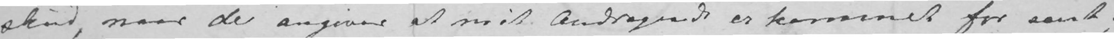skud, naar de angiver at mit Andragende er kommet for sent;
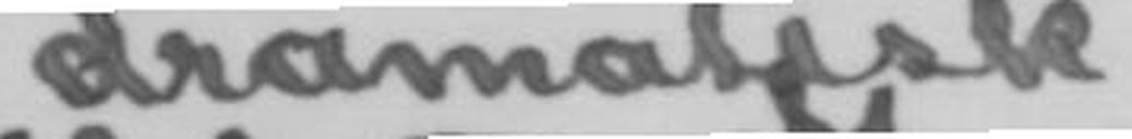dramatisk.
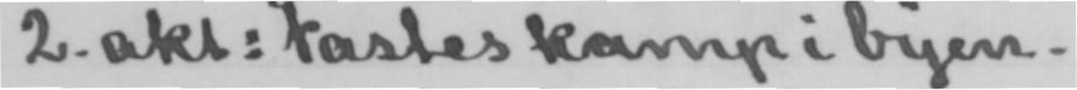2. akt: Fastes kamp i byen.
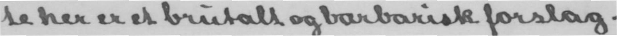te her er et brutalt og barbarisk forslag.
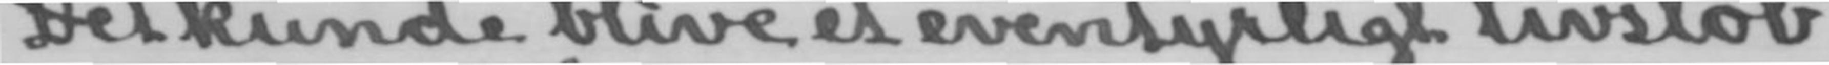Det kunde blive et eventyrligt livsløb
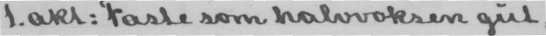1. akt: Faste som halvvoksen gut,
